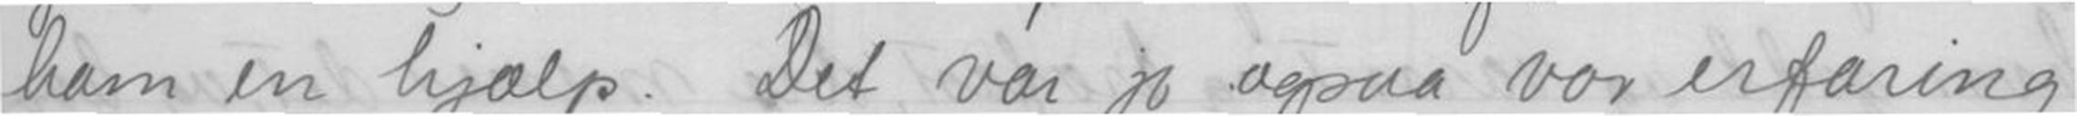ham en hjælp. Det var jo ogsaa vor erfaring
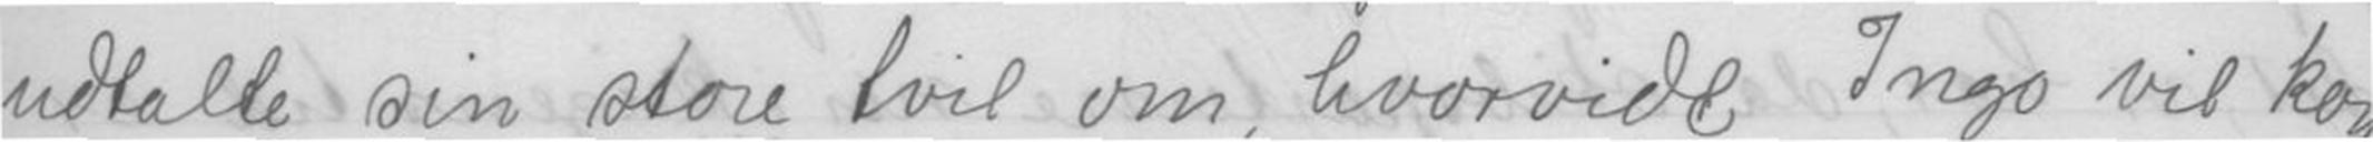udtalte sin store tvil om, hvorvide Ingo vil kom-
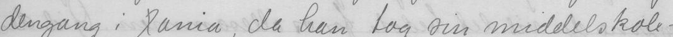dengang i Xania, da han tog sin middelskole-
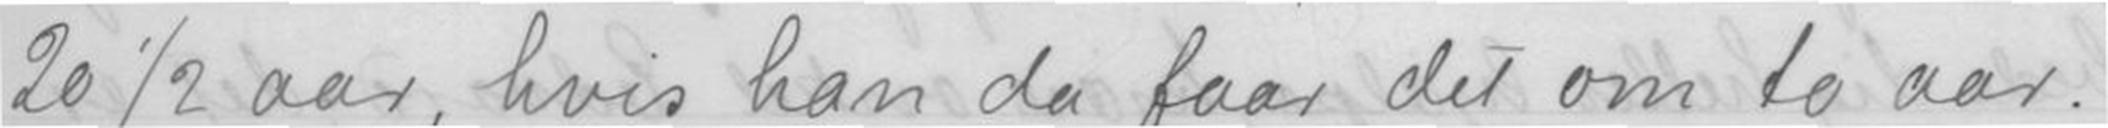20 1/2 aar, hvis han da faar det om to aar.
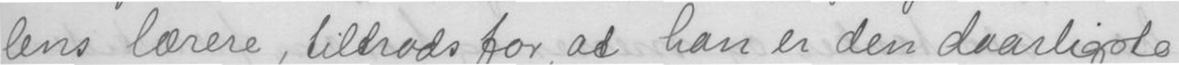lens lærere, tiltrods for, at han er den daarligste
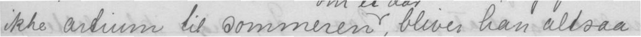ikke artium til sommeren, bliver han altsaa
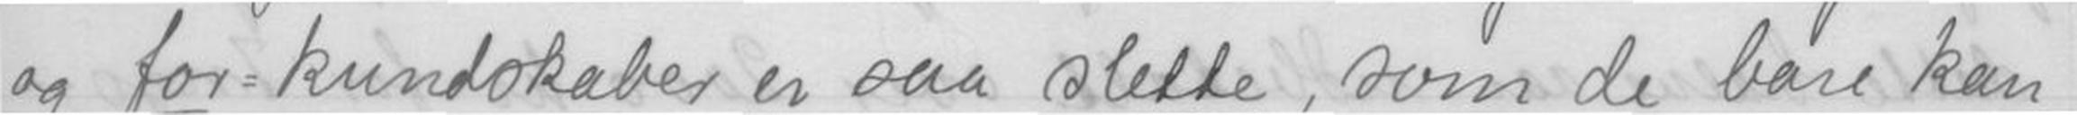og for=kundskaber er saa slette, som de bare kan
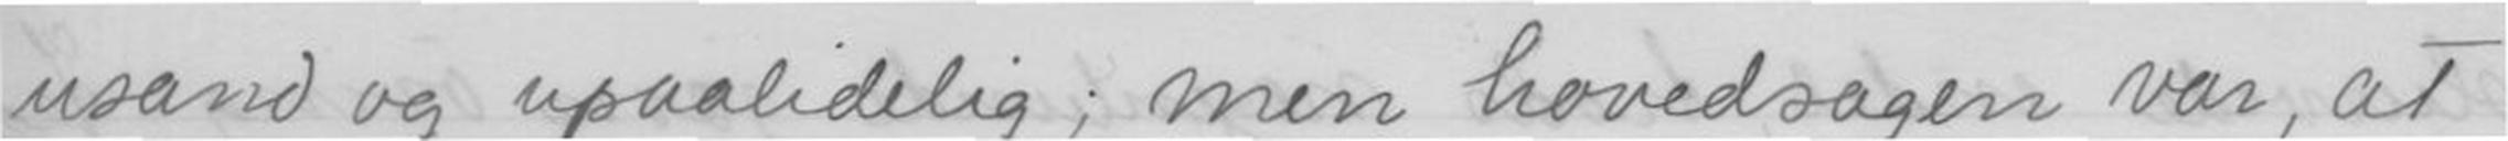usand og upaalidelig; men hovedsagen var, at
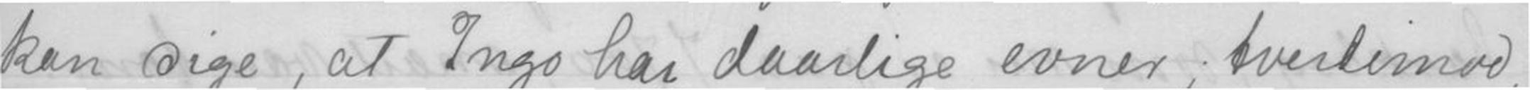kan sige, at Ingo har daarlige evner, tvertimod,
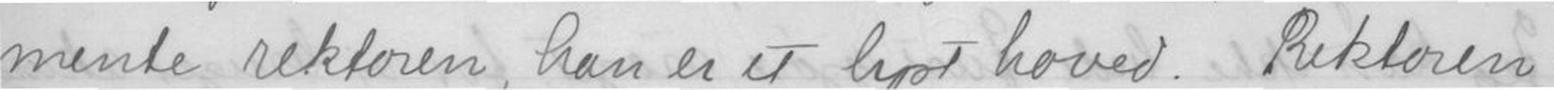mente rektoren, han er et lyst hoved. Rektoren
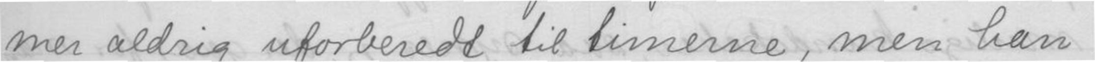mer aldrig uforberedt til timerne, men han
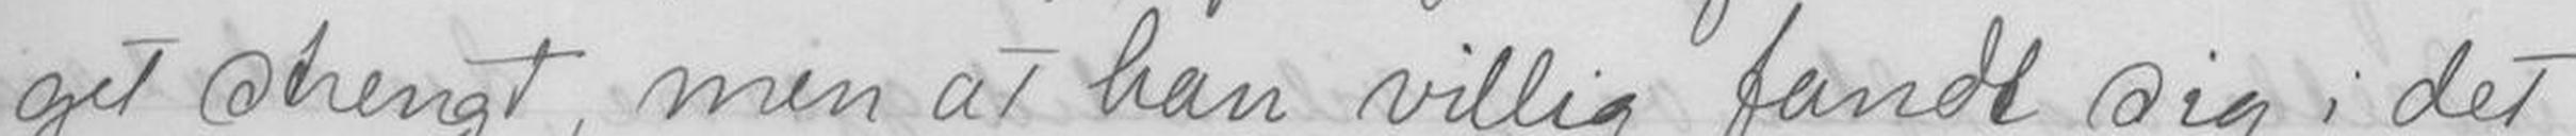get strengt, men at han villig fandt sig i det
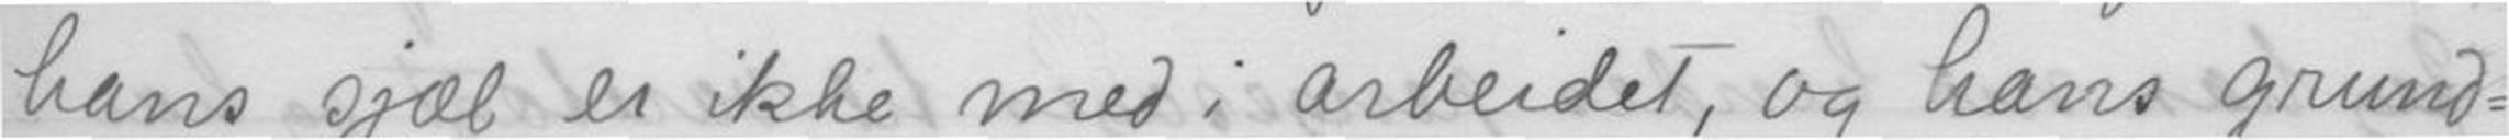hans sjæl er ikke med i arbeidet, og hans grund-
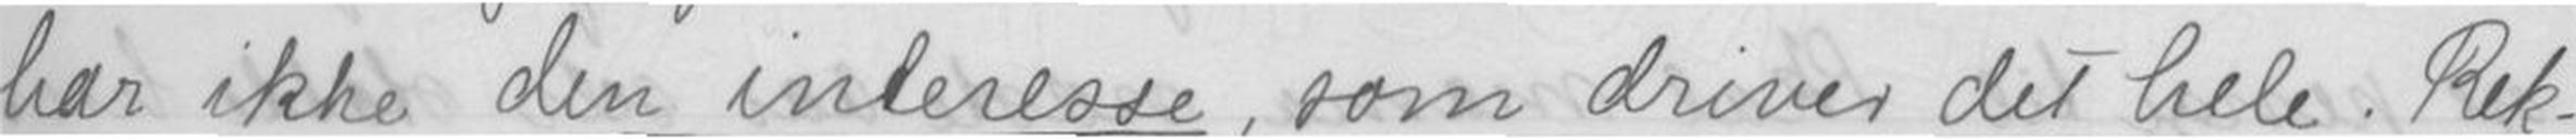har ikke den interesse, som driver det hele. Rek-
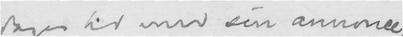dagers tid med sin annonce.
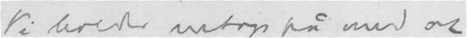Vi holder netop på med at
